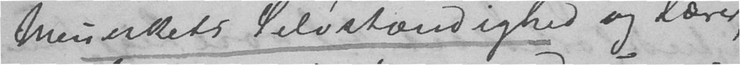Meneskets Selvstændighed og lærer,
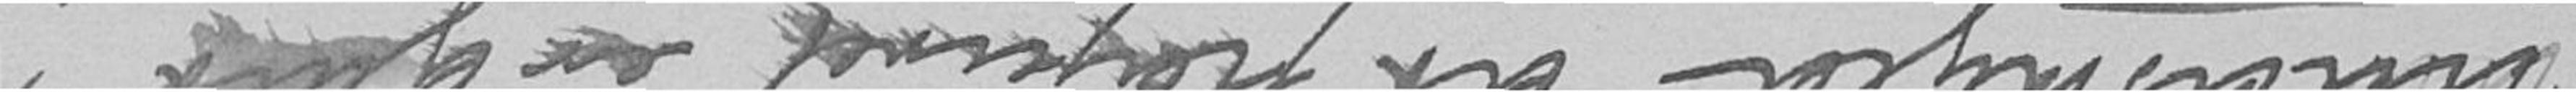Undskyld at papiret er gult!
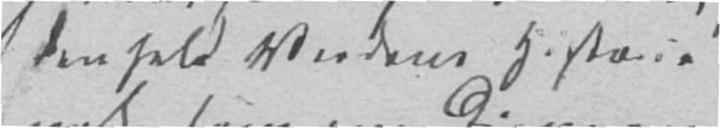den hele Verdens Historie
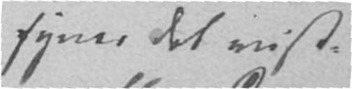synes det vist-
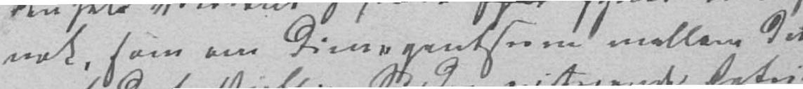nok, som om Divergentsen mellem de
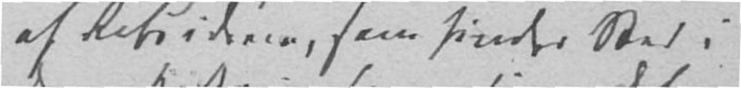af Retsideen, som finder Sted i
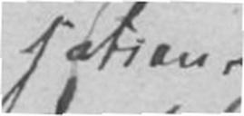sation
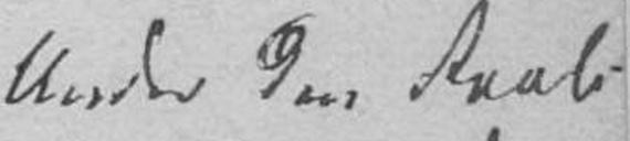Under den Reali-
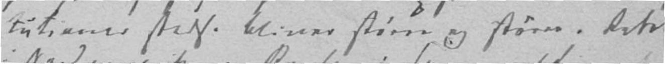tutioner stedse bliver større og større. Rets
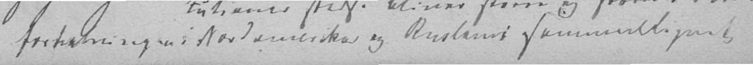forfatningen i Nordamerika og Rusland sammenlignet
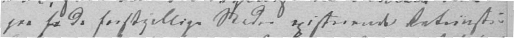paa de forskjellige Steder existerende Retsinsti-
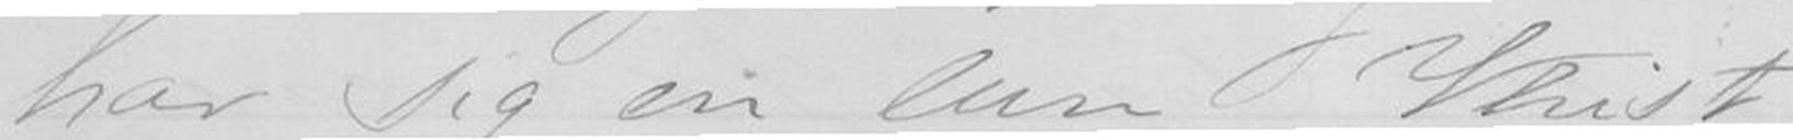har sig en lun Vhist
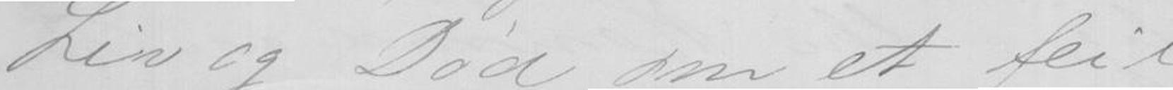Liv og Død om et feil
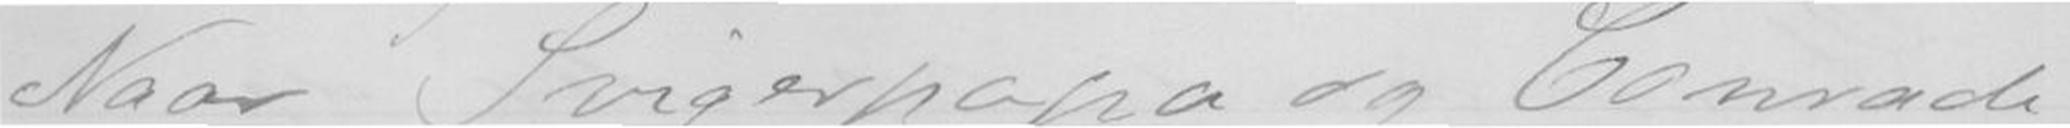Naar Svigerpapa og Conradi
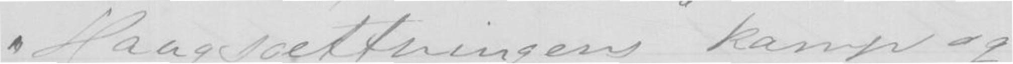Haagsættningens kamp og
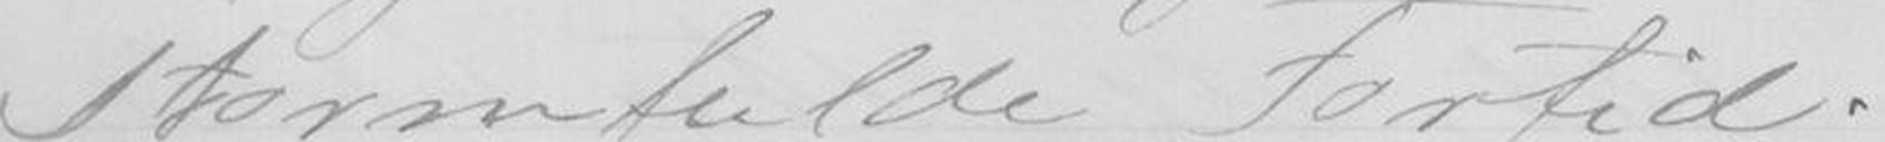stormfulde Fortid. -
